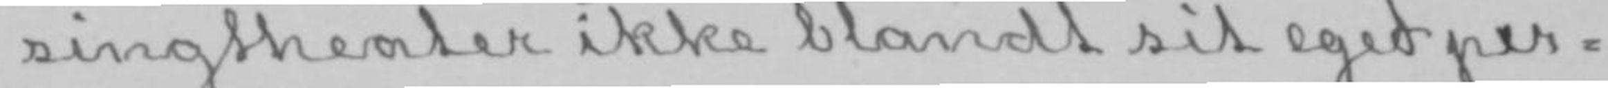singtheater ikke blandt sit eget per-
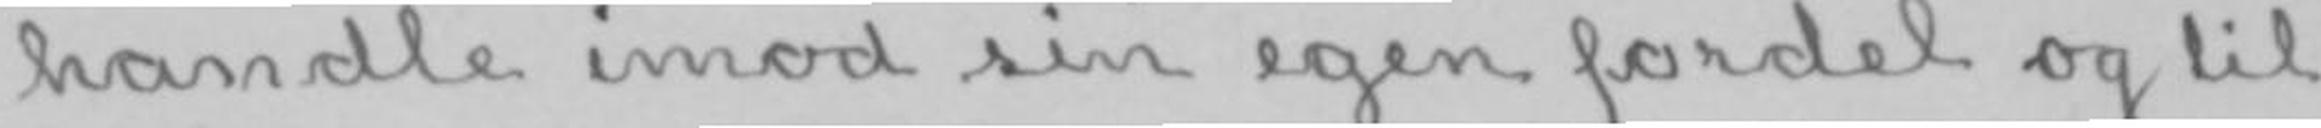handle imod sin egen fordel og til
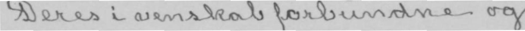Deres i venskab forbundne og
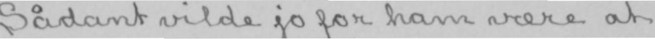Sådant vilde jo for ham være at
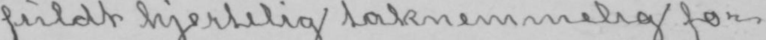fuldt hjertelig taknemmelig for
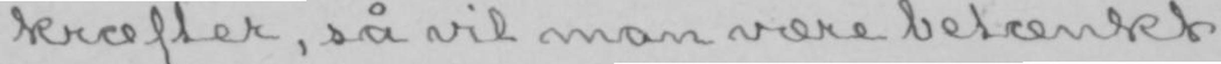kræfter, så vil man være betænkt
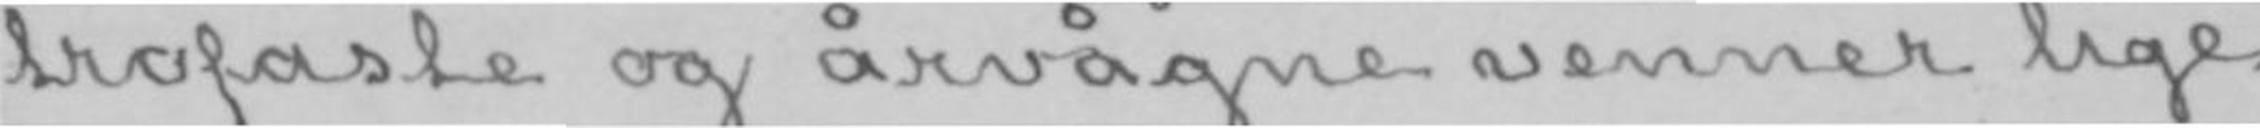trofaste og årvågne venner lige
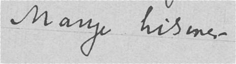Mange hilsener
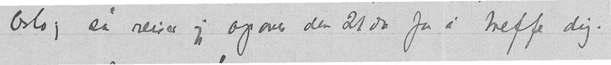Oslo, så reiser jeg opover den 21de for å treffe dig.
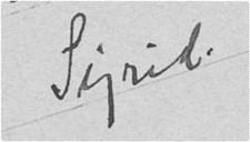Sigrid.
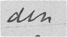din
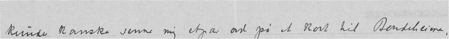kunde kanske senne mig etpar ord på et kort til Bondeheimen,
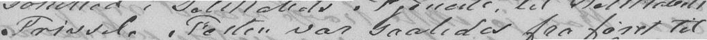Trivsel Festen var saaledes for først til
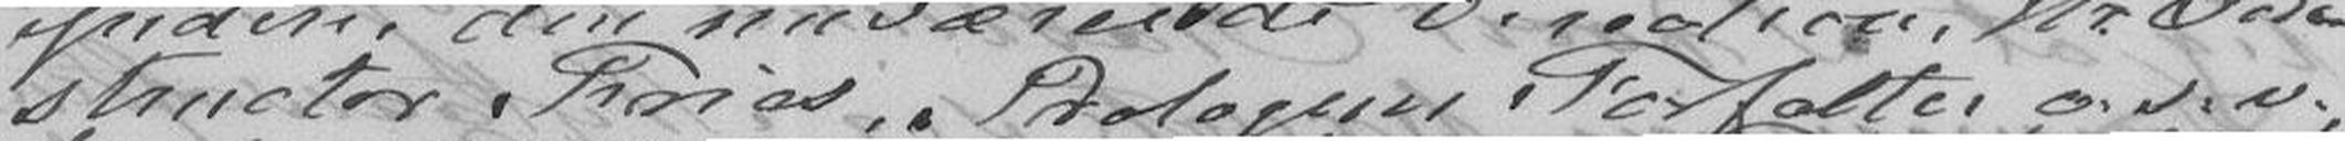structor Fries, Prologens Forfatter o.s.v.
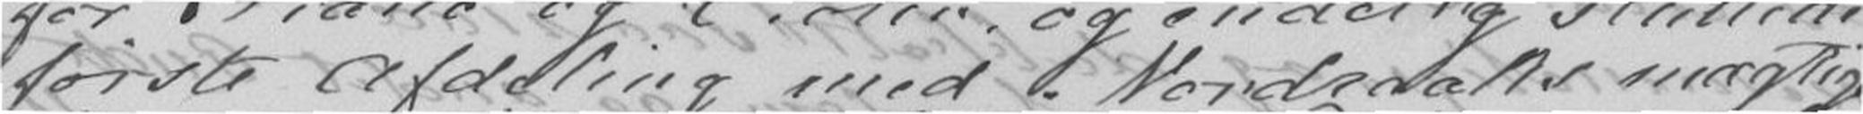første Afdeling med Nordraaks mægtige
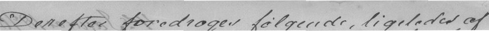Derefter foredrager følgende, ligeledes af
